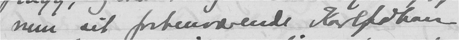nem sit forhenværende KarlJohan
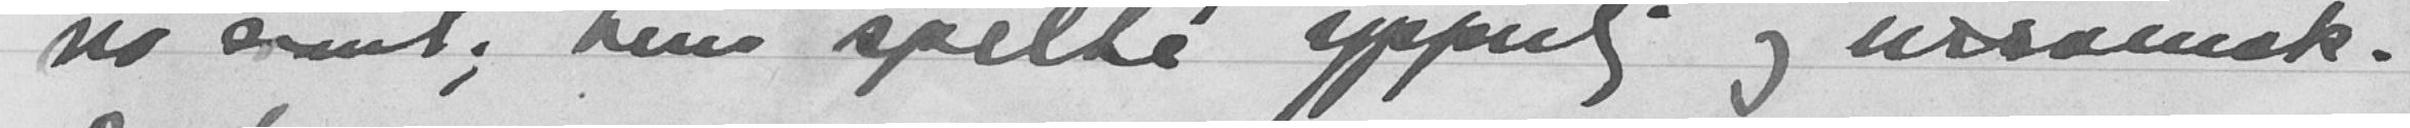no saant; hun spilte ypperlig og ursvensk.
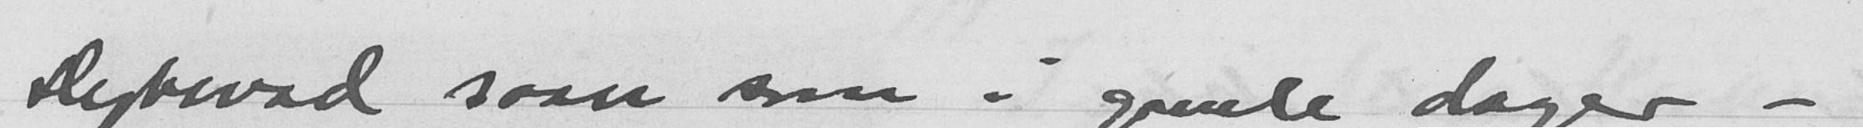Dybwad saan som i gamle dager -
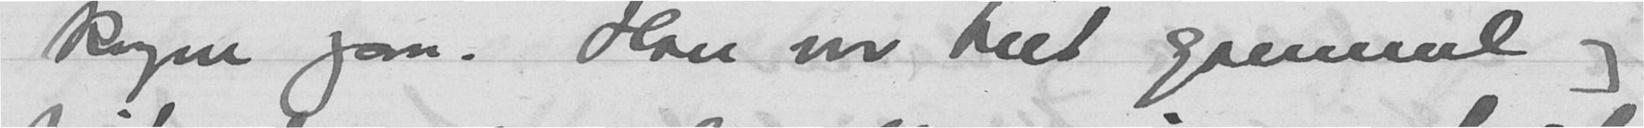kongen Jon. Han var blit gammel og
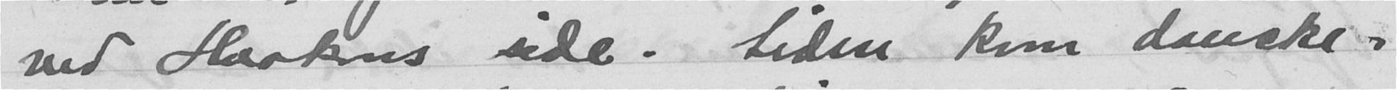ved Haakons side. Siden kom danske-
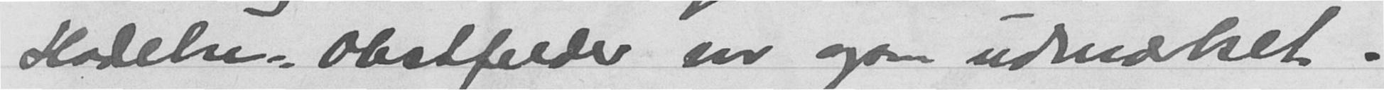Hodebu - Obstfelder var ogsaa udmerket.
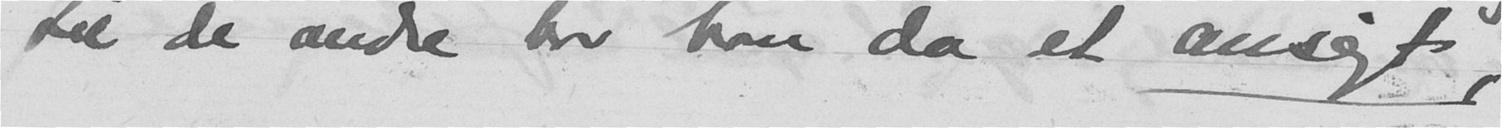til de andre har han da et ansigt,
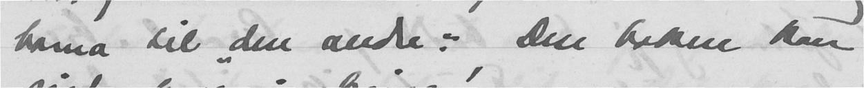barna til "den andre". Den boken kan
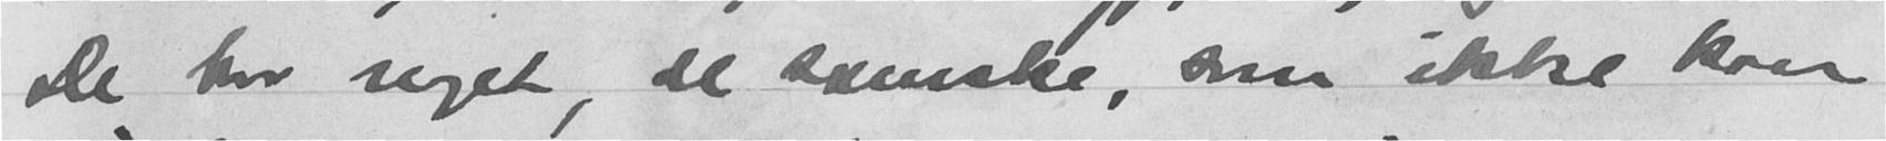De har noget, de svenske, som ikke kan
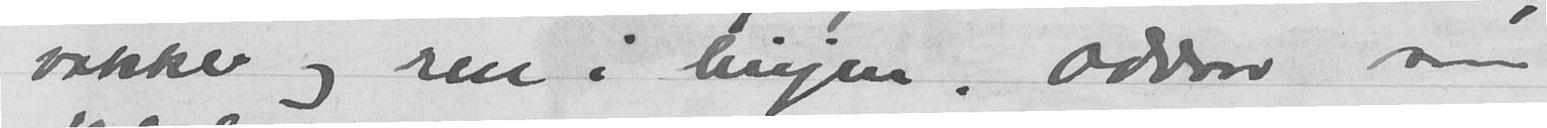vakker og ren i linjen. Odden var
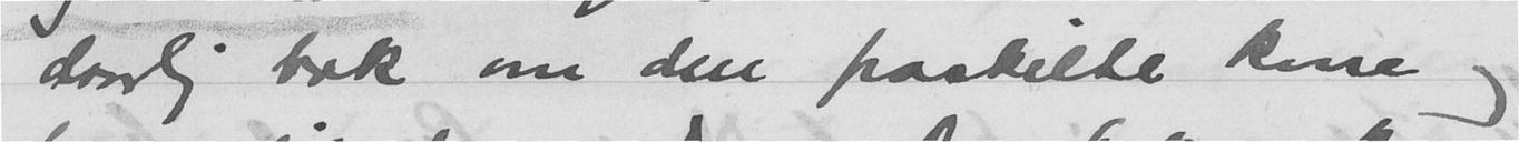daarlig bok om den fraskilte kone og
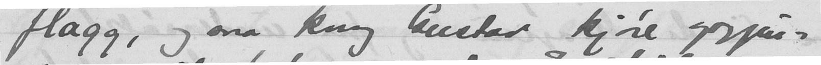flagg, og saa kong Gustav kjøre opgjen-
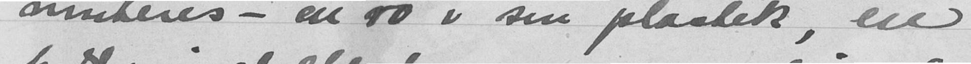imiteres - en ro i sin plastik, en
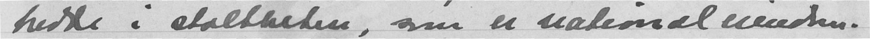bredde i stoltheten, som er nationaleiendom.
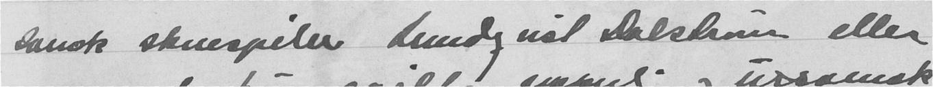svensk skuespiler Lundquist Dalstrøm eller
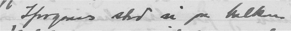Iforgaars stod vi på balkonen
